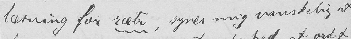læsning for rætr, synes mig vanskelig at
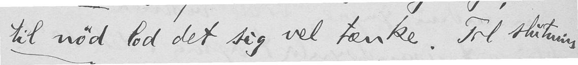til nød lod det sig vel tænke. Til slutning
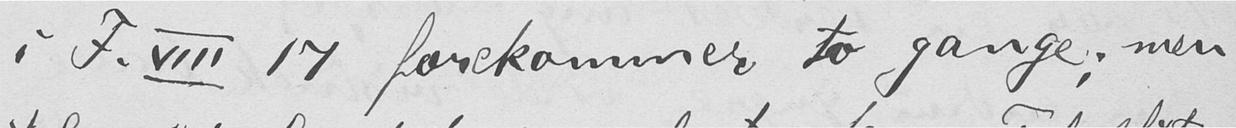i F. VIII 17 forekommer to gange; men
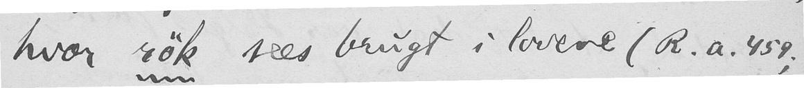hvor rök sees brugt i lovene (R. a. 459;
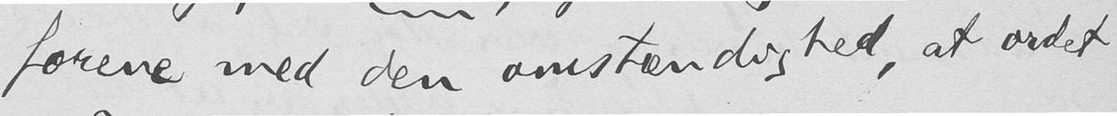forene med den omstændighed, at ordet
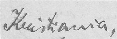Kristiania,
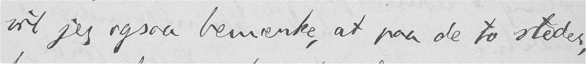vil jeg ogsaa bemærke, at paa de to steder,
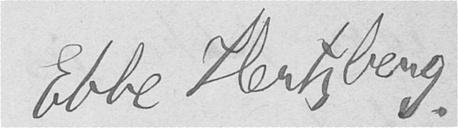Ebbe Hertzberg.
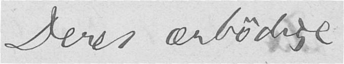Deres ærbødige
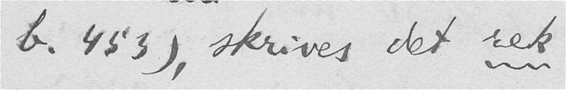b. 453), skrives det rek.
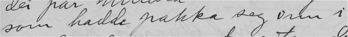som hadde pakka seg inn i
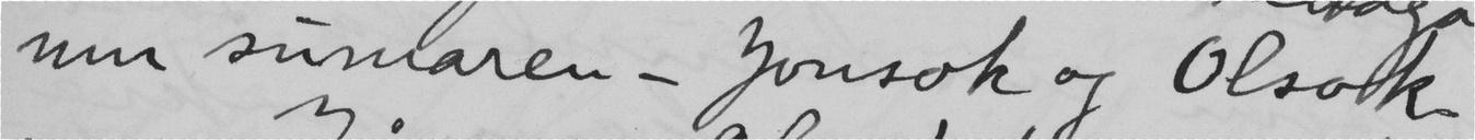um sumaren – jonsok og Olsok.
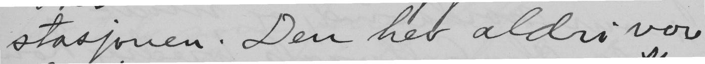stasjonen. Den hev aldri vore
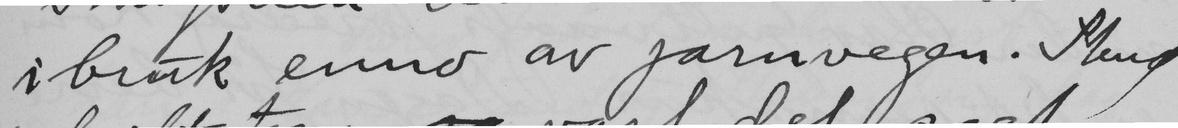ibruk enno av jarnvegen. Stend
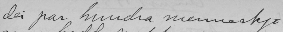dei par hundra menneskje
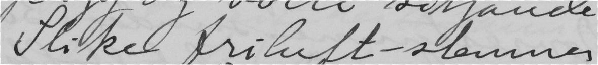Slike friluft-stemner
